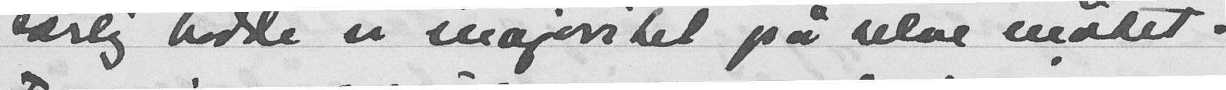særlig hadde vi majoritet på selve møtet.
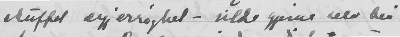skuffet ærgjerrighet - vilde gjerne selv bli
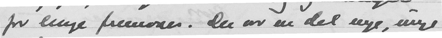for lenge fremover. Der var en del nye, unge
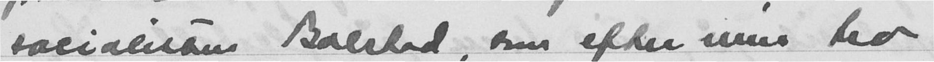socialisten Balstad, som efter min tro
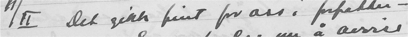11/11 Det gikk fint for oss i forfatter-
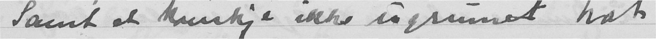Samt et kanskje ikke ubegrunnet hat
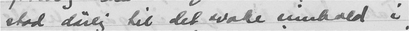stod dårlig til det svake innhold i
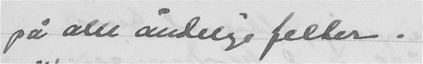på alle åndelige felter!
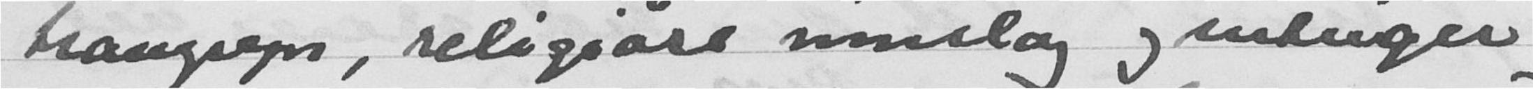trangsyn, religiøse innslag og intriger
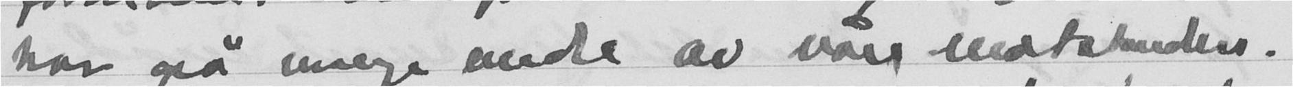har også mange andre av våre motehunder.
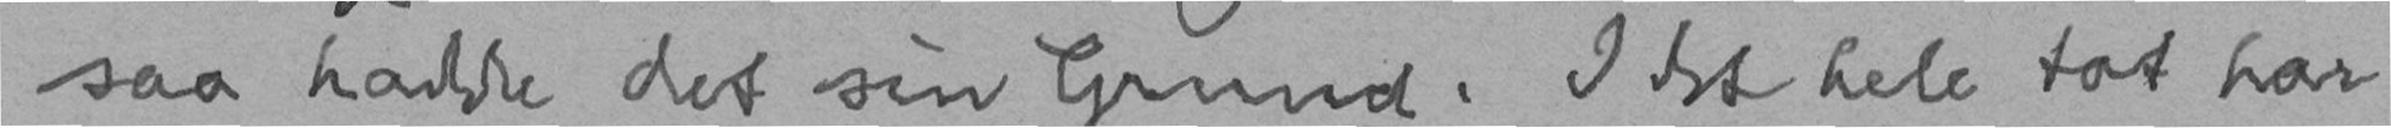saa hadde det sin Grund. I det hele tat har
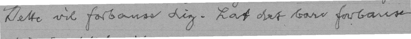Dette vil forbause dig. Lat det bare forbause
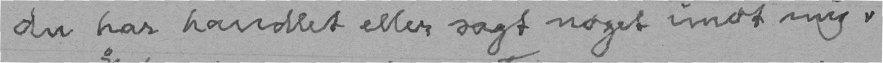du har handlet eller sagt noget imot mig.
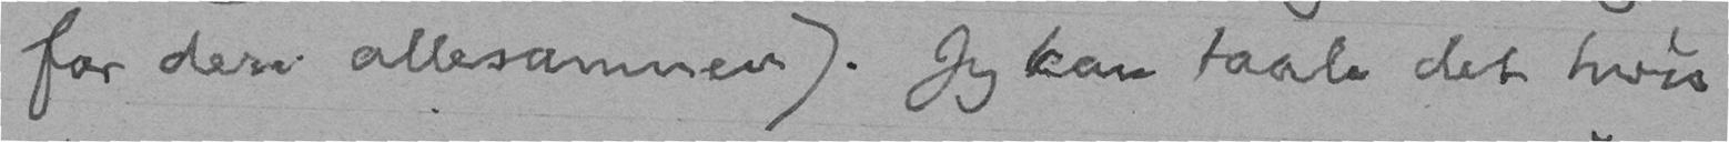for dere allesammen). Jeg kan taale det hvis
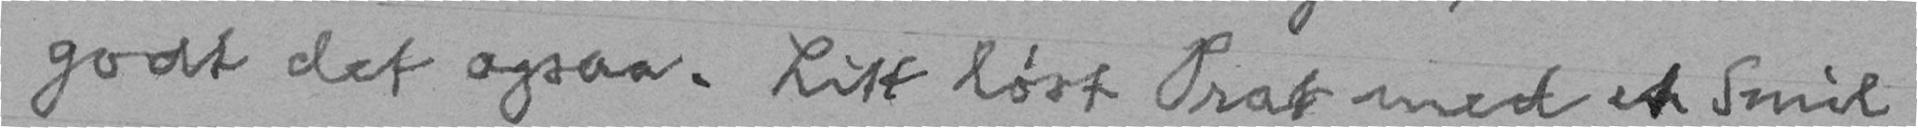godt det ogsaa. Litt løst Prat med et Smil
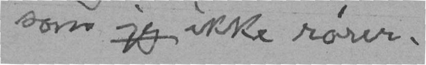som jeg ikke rører.
